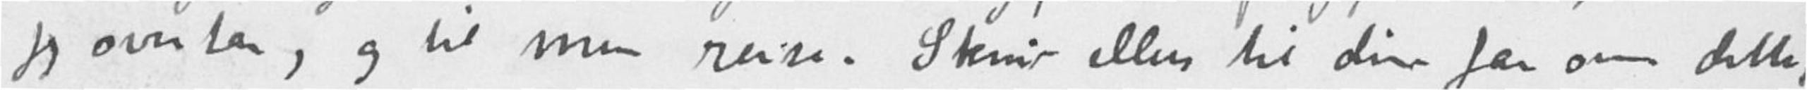jeg overtar, og til min reise. Skriv ellers til din far om dette.
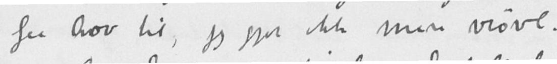faa lov til, jeg gjør ikke mere vrøvl.
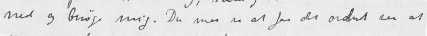ned og besøge mig. Du maa se at faa det ordnet saa at
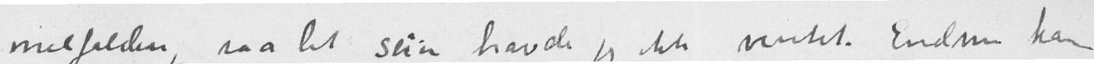melfalden, saa let seier havde jeg ikke ventet. Endnu kan
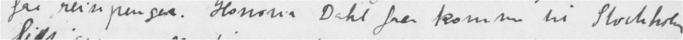faa reisepenger. Honoria Dahl faar komme til Stockholm
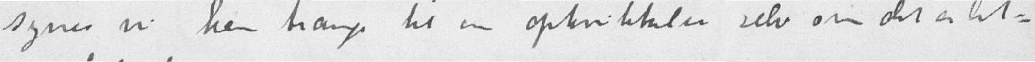synes vi kan trænge til en opkvikkelse selv om det er let-
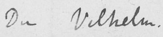Din Vilhelm.
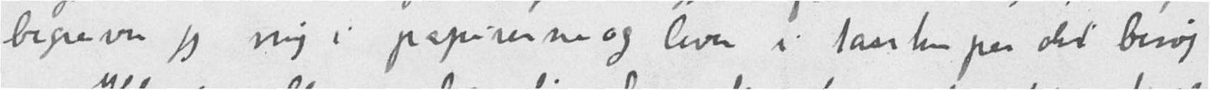begraver jeg mig i papirerne og lever i tanken paa dit besøg
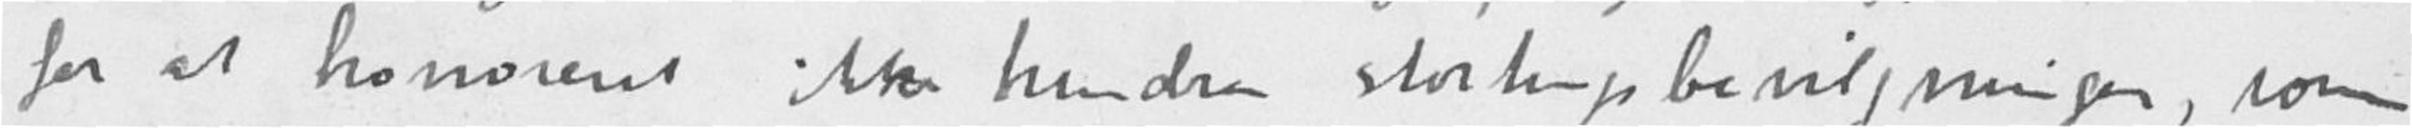for at honoraret ikke hindrer stortingsbevilgningen, som
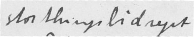storthingsbidraget
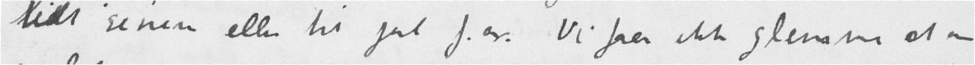lidt senere eller til jul f.ex. Vi faar ikke glemme at en
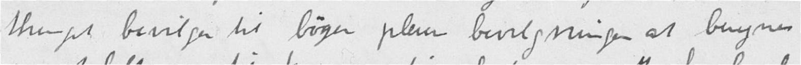thinget bevilger til bøger pleier bevilgningen at beregnes
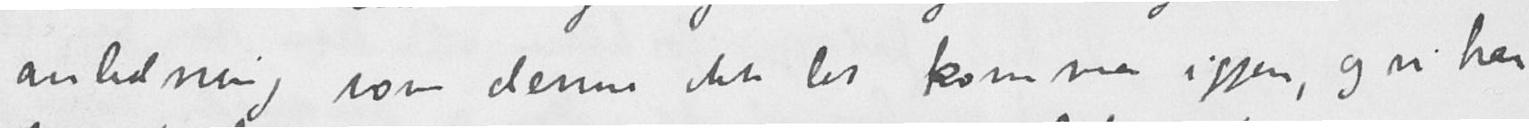anledning som denne ikke let kommer igjen, og vi har
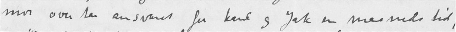mor overtar ansvaret for Karl og Jak en maaneds tid,
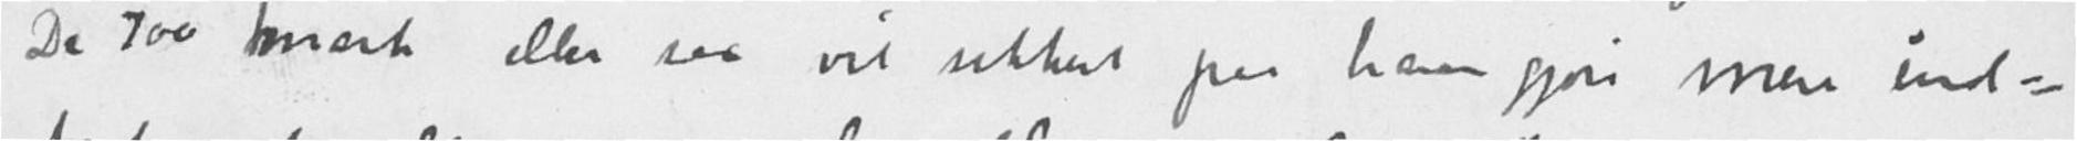De 700 mark eller saa vil sikkert paa ham gjøre mere ind-
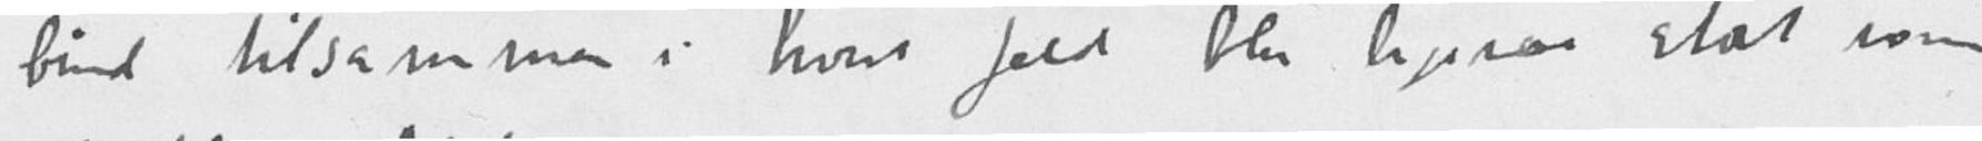bind tilsammen i hvert fald blir ligesaa stort som
